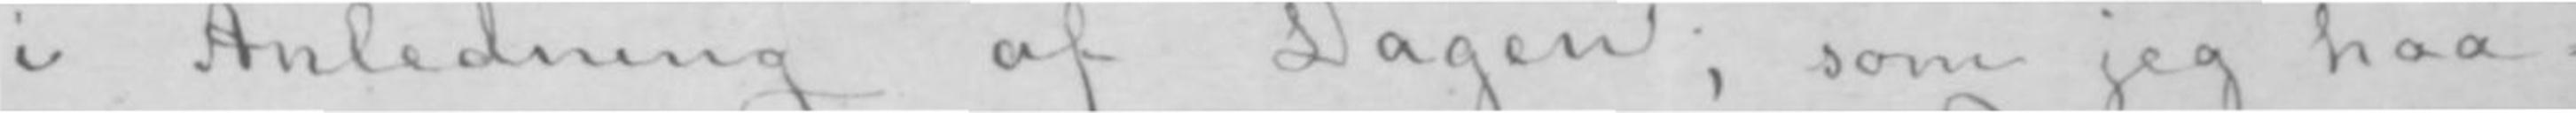i Anledning af Dagen; som jeg haa-
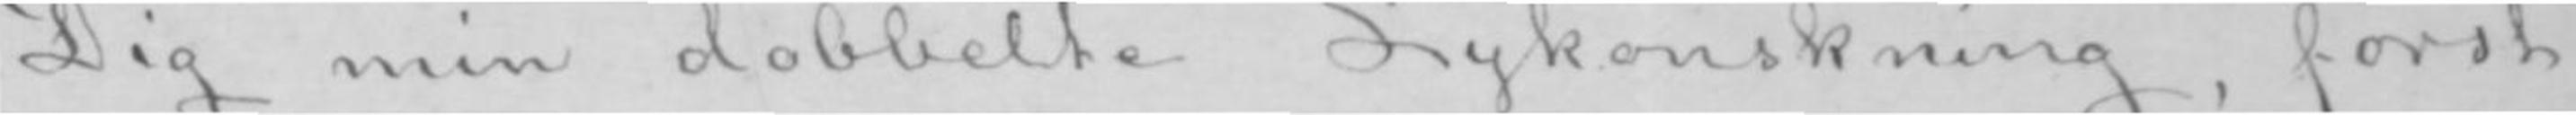Dig min dobbelte Lykønskning, først
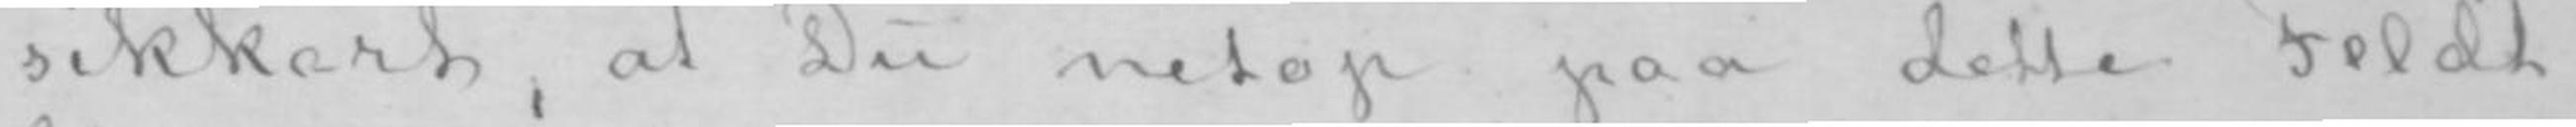sikkert, at Du netop paa dette Feldt
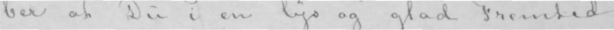ber at Du i en lys og glad Fremtid
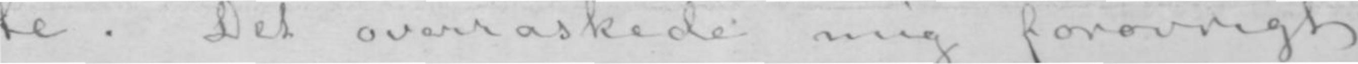te. Det overraskede mig forøvrigt
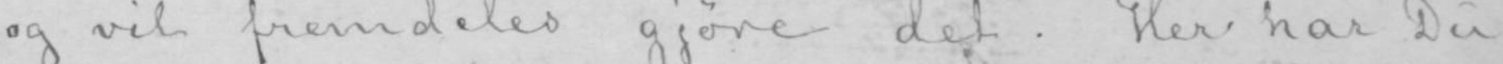og vil fremdeles gjøre det. Her har Du
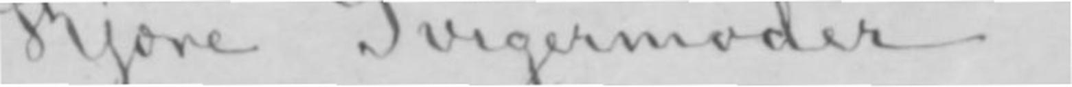Kjære Svigermoder!
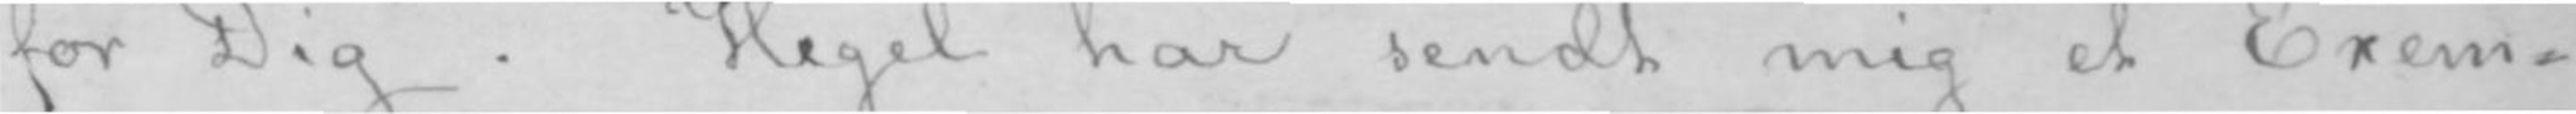for Dig. Hegel har sendt mig et Exem-
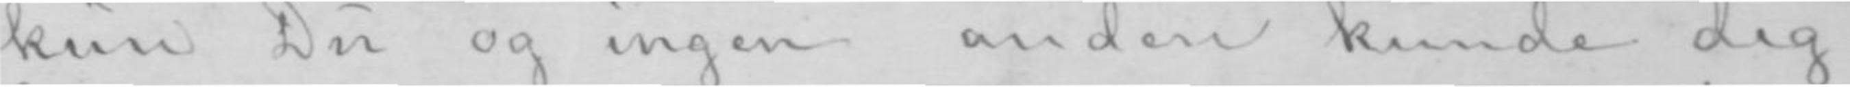kun Du og ingen anden kunde dig-
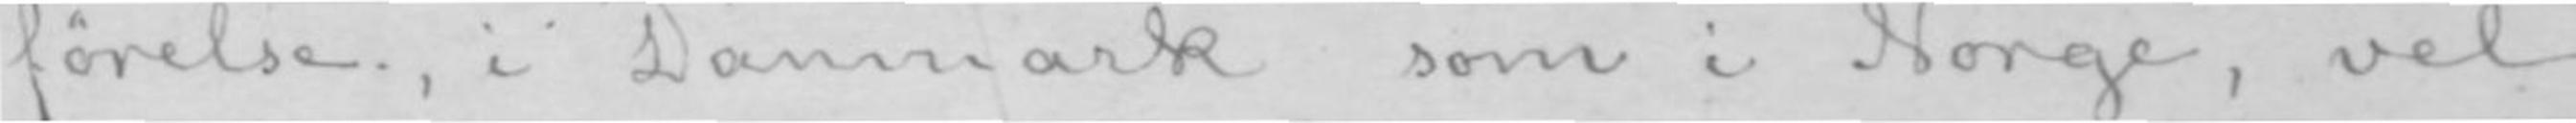førelse, i Danmark som i Norge, vel
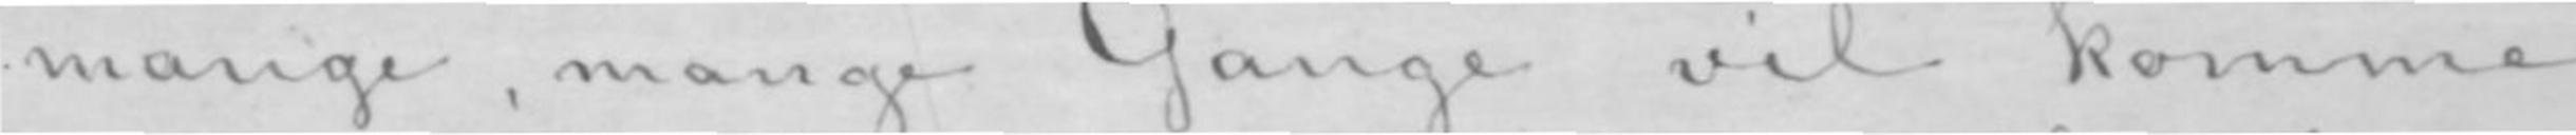mange, mange Gange vil komme
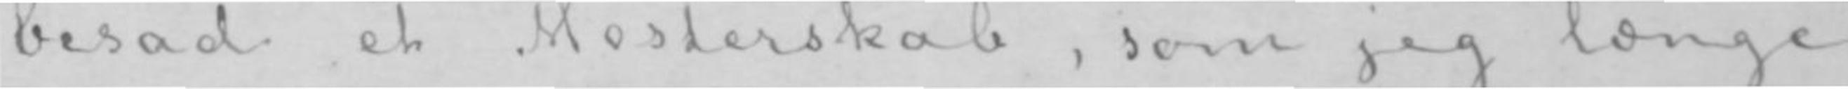besad et Mesterskab, som jeg længe
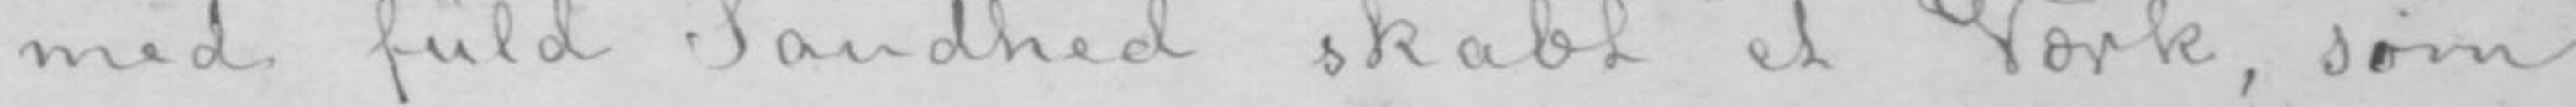med fuld Sandhed skabt et Værk, som
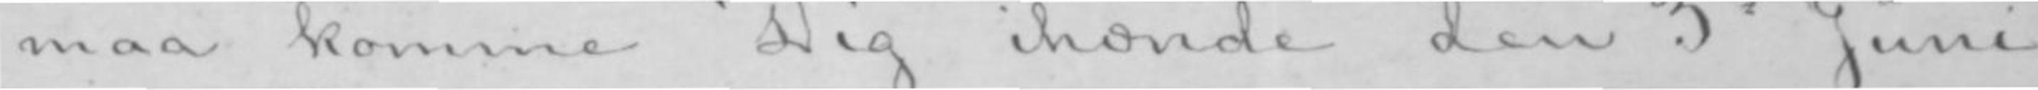maa komme Dig ihænde den 3die Juni
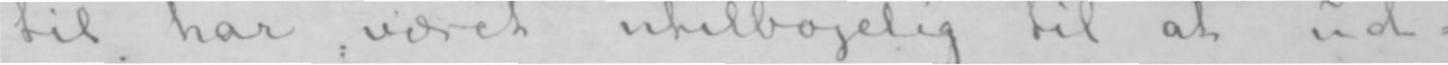til har været utilbøjelig til at ud-
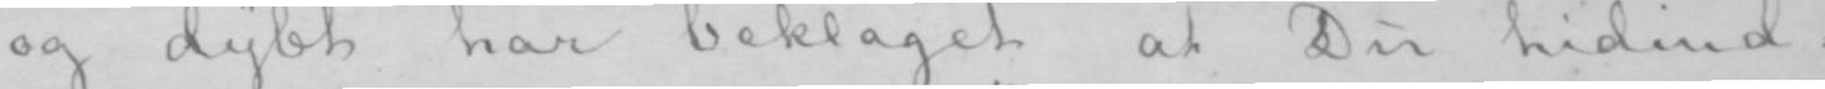og dybt har beklaget at Du hidind-
